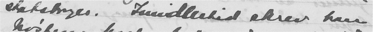statsborger. Imidlerted skrev han
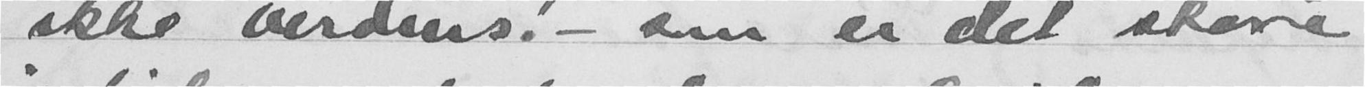ikke verdens" - som er det store
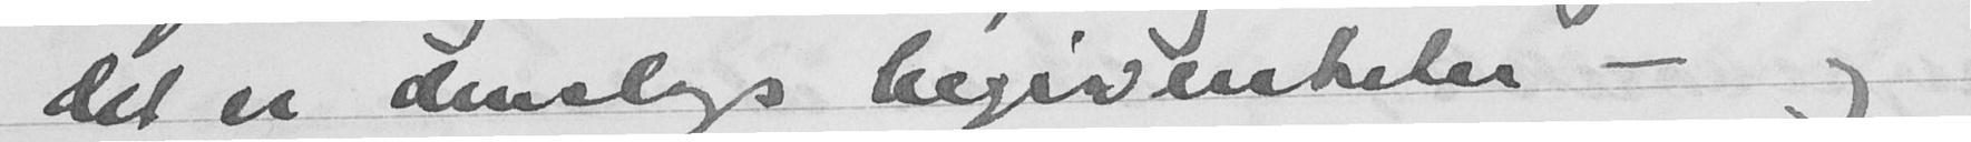det er denslags begivenheter - og
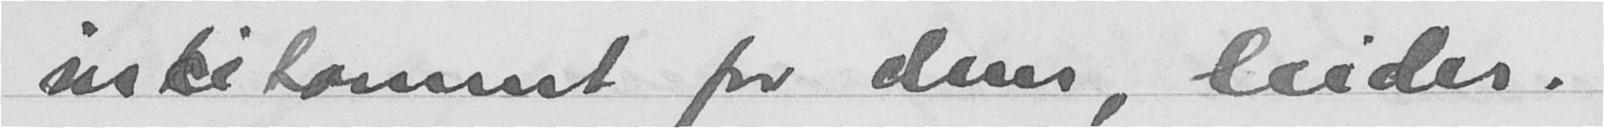uskitommt for dem, leides.
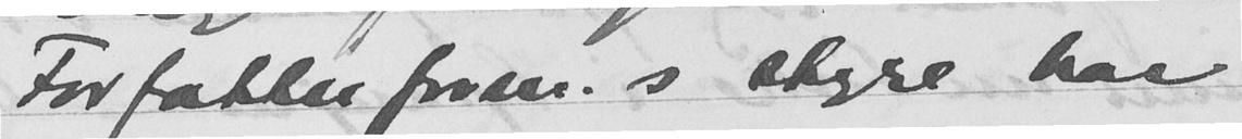Forfatterforen.s styre har
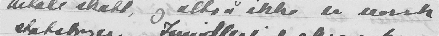betale skatt, og altså ikke er norsk
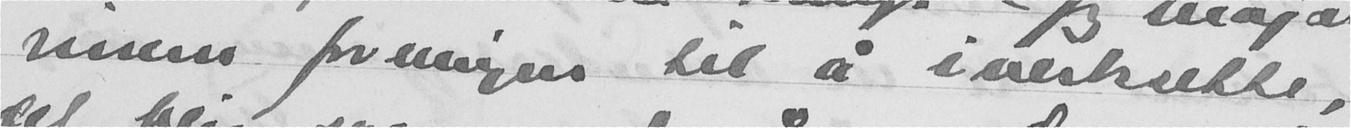innen foreningen til å iverksette,
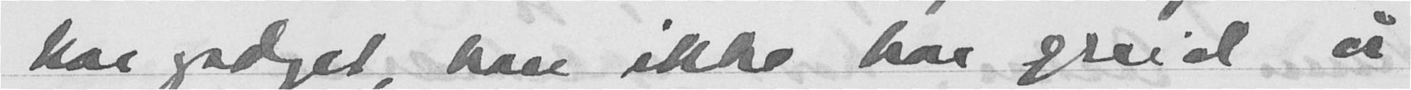har opdaget, han ikke har greid å
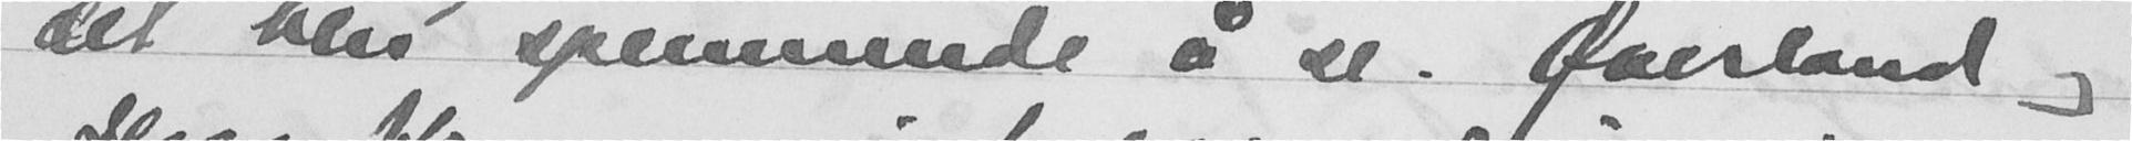det blir spennende å se. Øverland og
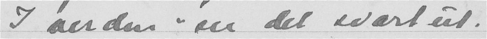I verden ser det svart ut.
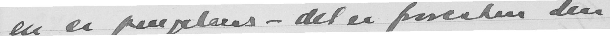en er pengelens - det er forresten den
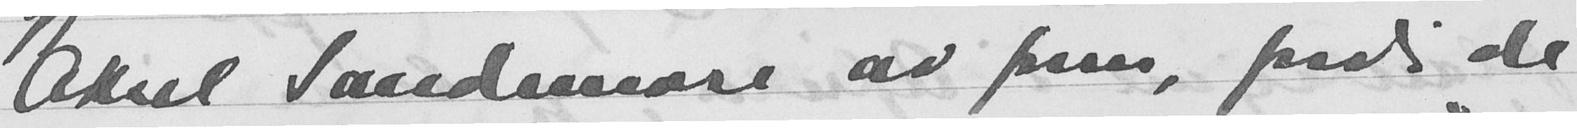Aksel Sandemose av foren, fordi de
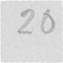20
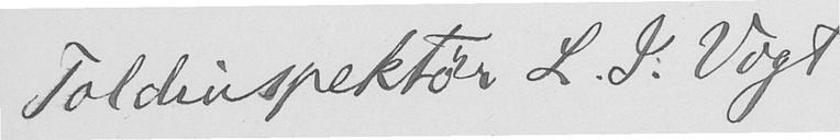Toldinspektør L. J. Vogt
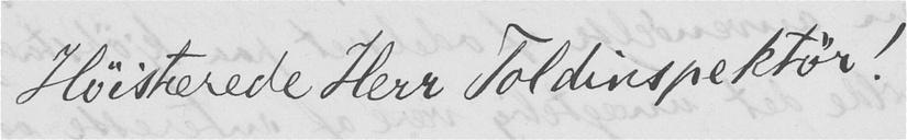Høistærede Herr Toldinspektør!
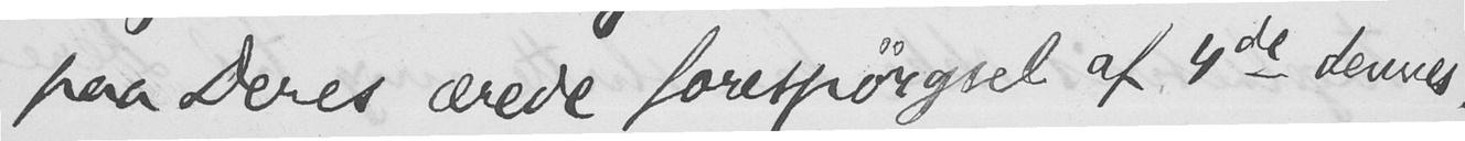paa Deres ærede forespørgsel af 4de dennes.
